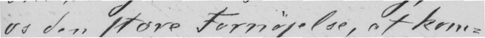os den store Fornøjelse, at kom-
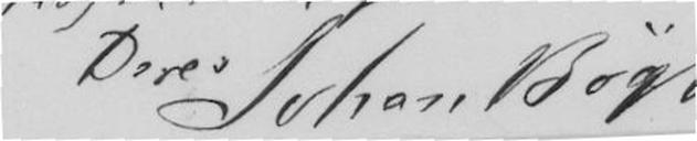Deres Johan Bøgh
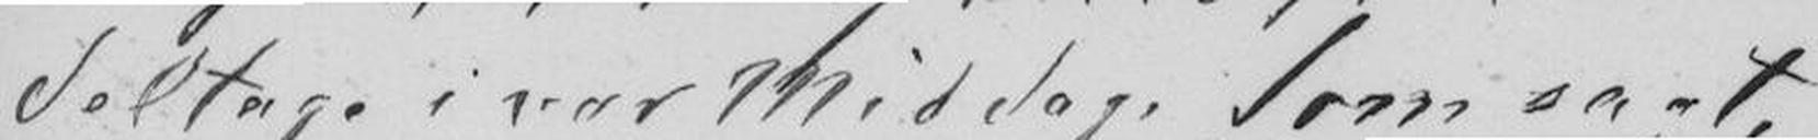deltage i vor Middag. Som sagt
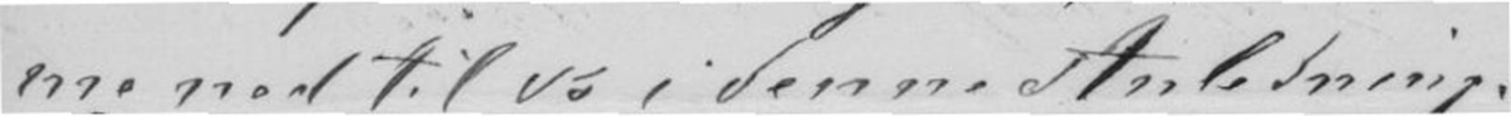me ned til os i denne Anledning.
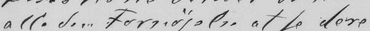alle den Fornøjelse at se dere.
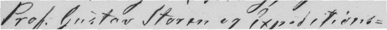Prof. Gustav Storm og Expeditions-
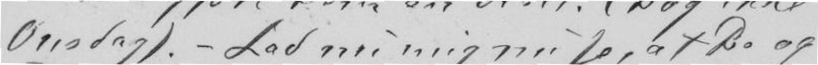Onsdag). - Lad nu mig nu se, at De og
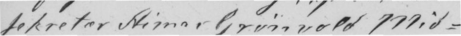sekretær Aimar Grønvold Mid-
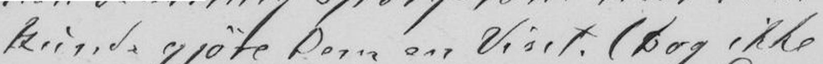kunde gjøre Dem en Visit. (Dog ikke
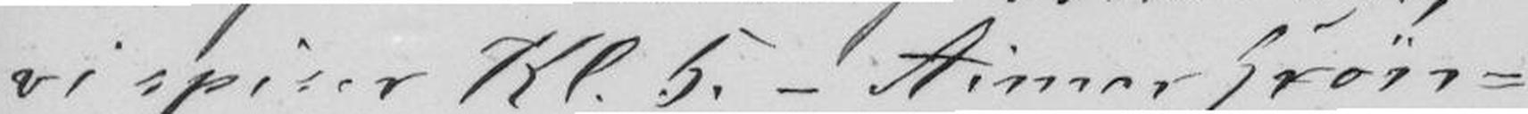vi spiser Kl. 5. - Aimar Grøn-
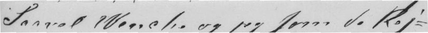Saavel Wenche og jeg som de Rej-
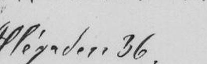Allegaden 36.
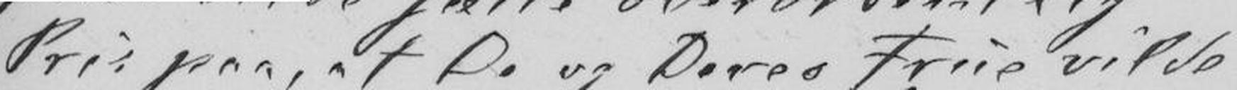Pris paa, at De og Deres Frue vilde
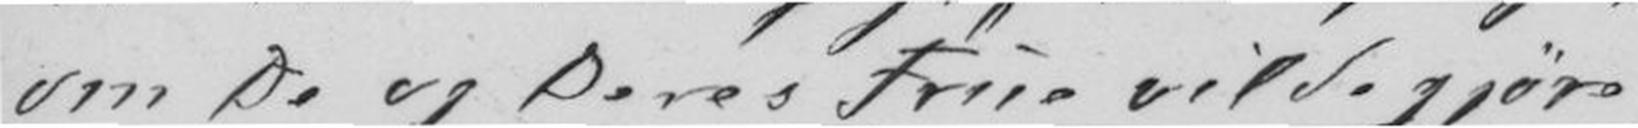om De og Deres Frue vilde gjøre
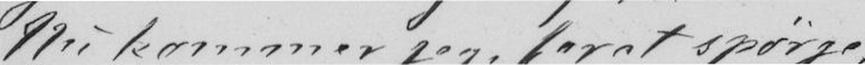Nu kommer jeg, forat spørge
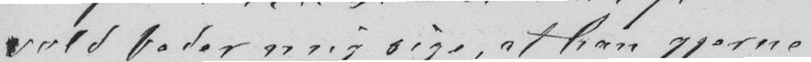vold beder mig sige, at han gjerne
